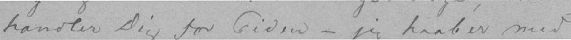handler Dig for Giden - jeg haaber med
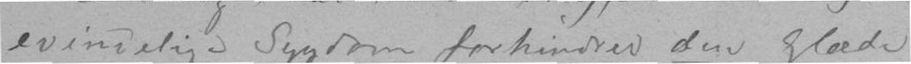evindelige Sygdom forhindrer den glæde
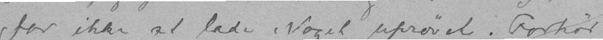for ikke at lade Nogel uprøvel. Godhør
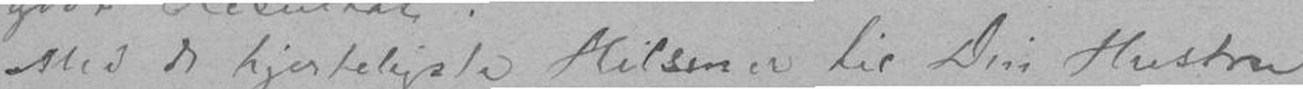Med de hjerteligste Hilsener til Din Hustru
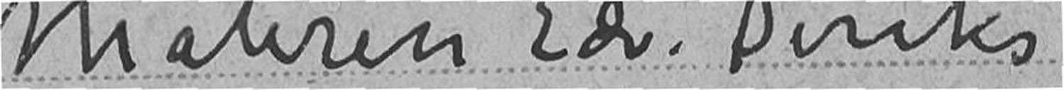Maleren Edv. Diriks
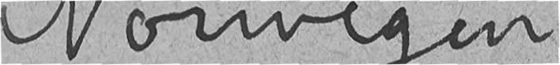Norwegen
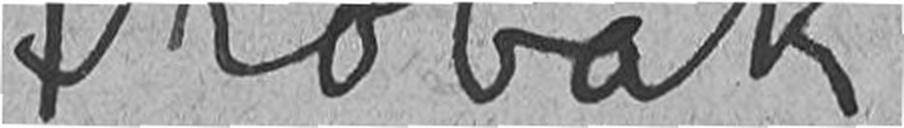Drøbak
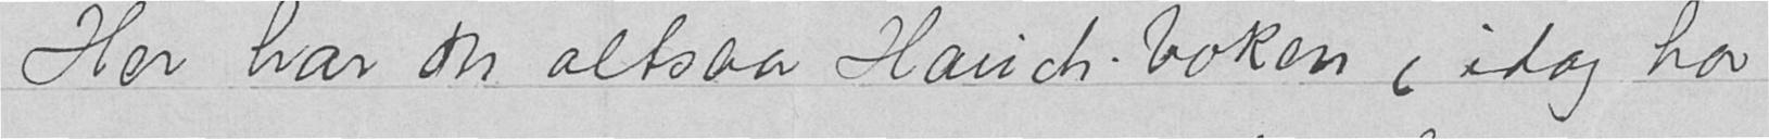Her har du altsaa Hauch-boken (idag har
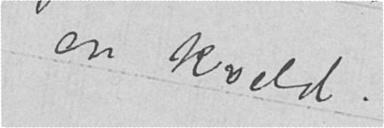en kveld.
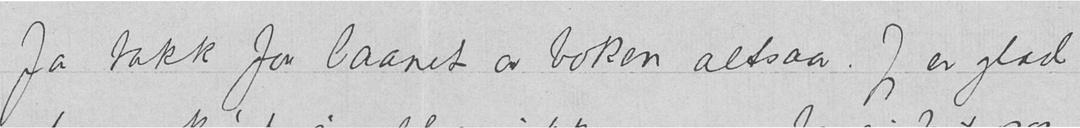Ja takk for laanet av boken altsaa. Jeg er glad
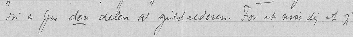du er for den delen av guldalderen. For at vise dig at jeg
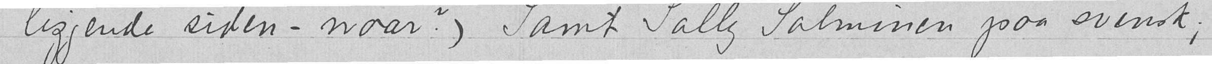liggende siden – naar?) Samt Sally Salminen paa svensk;
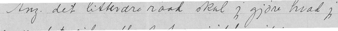Ang. det litterære raad skal jeg gjøre hvad jeg
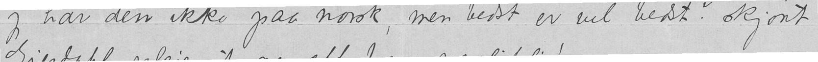jeg har den ikke paa norsk, men bedst er vel bedst? skjønt
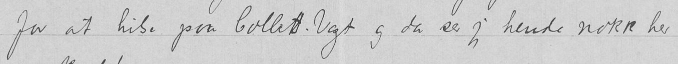for at hilse paa Collett-Vogt og da ser jeg hende nokk her
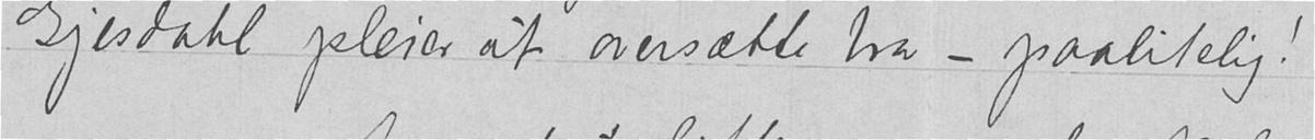Gjesdahl pleier at oversætte bra – paalitelig!
